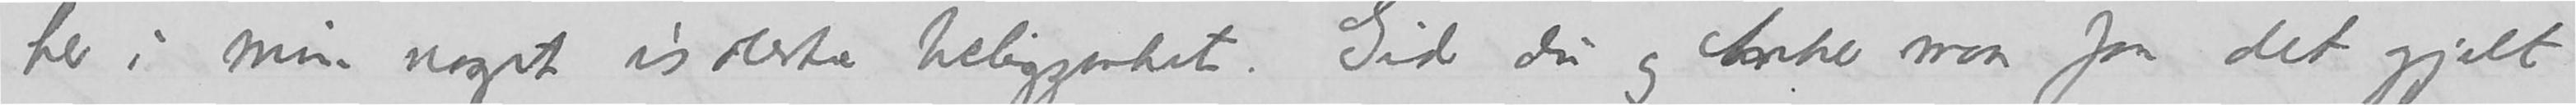her i min noget isolerte beliggenhet. Gid du og Anker mon faar det gjilt
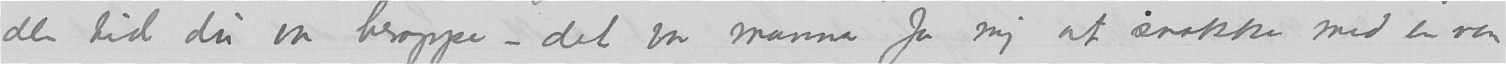den tid du var heroppe – det vaer manna for mig at snakke med en ven
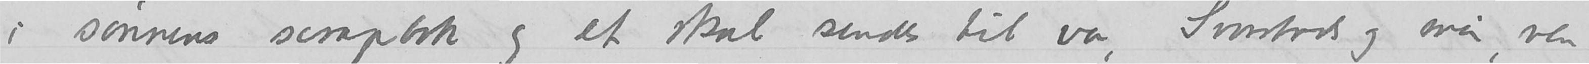i sønnens scrapbok og et skal sendes til vaar, Svarstads og min, ven pr.
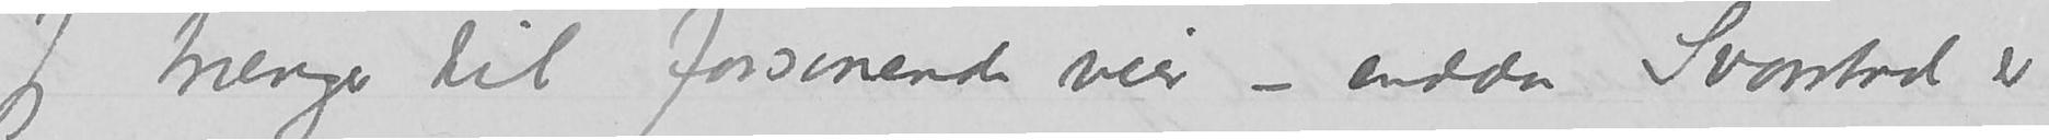Jeg trenger til forsonende veir - endda Svarstad er
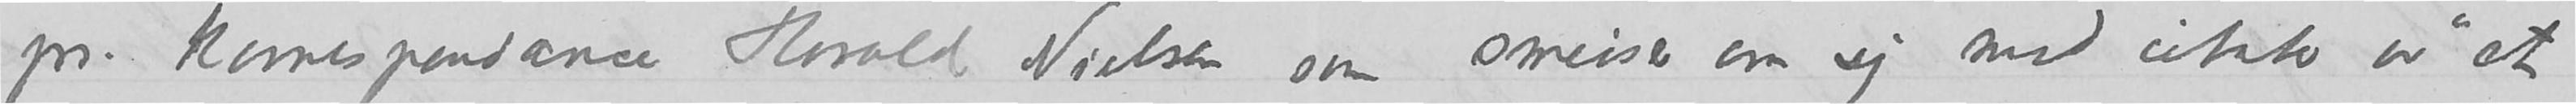korrepondance Harald Nielsen, som smeiser om sig med citater av «et
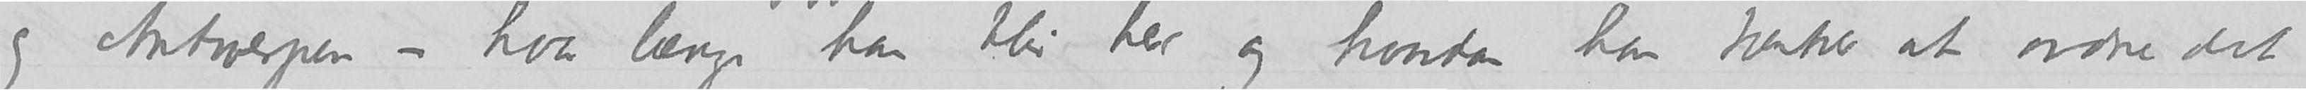og Antwerpen - hvor længe han blir her og hvordan han tænker at ordne det
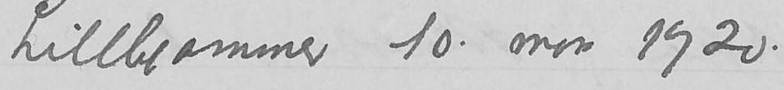Lillehammer 10. mars 1920.
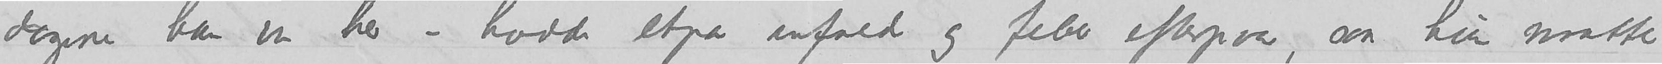dagene han var her - hadde etpar anfald og feber efterpaa, saa hun maatte
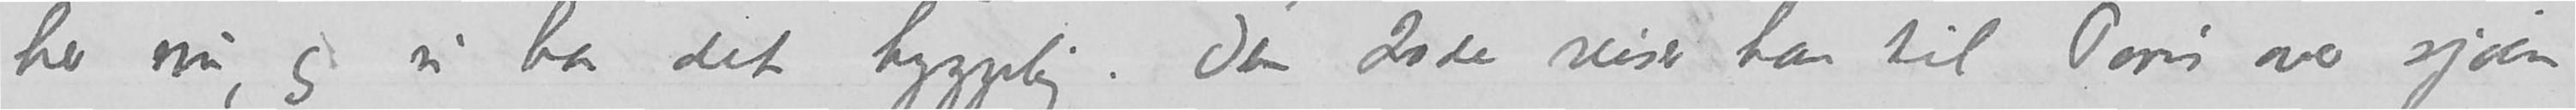her nu, og vi har det hyggelig. Den 20de reiser han til Paris over sjøen
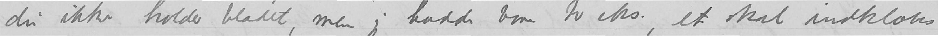du ikke holder bladet, men jeg hadde bare to eks, et skal indklæbes
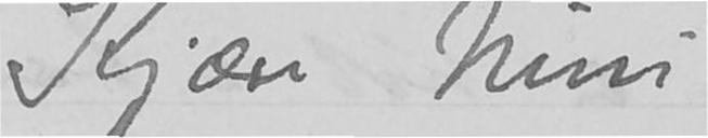Kjære Nini
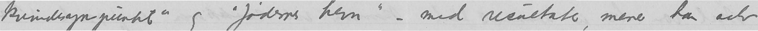Kvindesynspunkt» og «Jjødernes hevn» –- med resultater, mener han selv
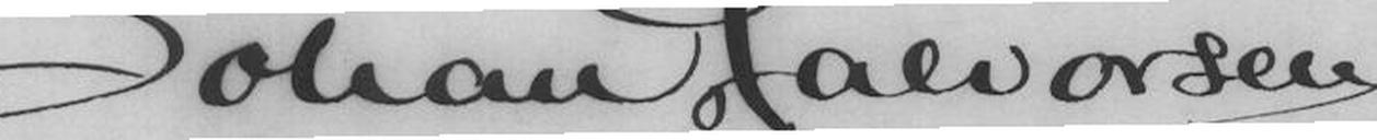Johan Halvorsen
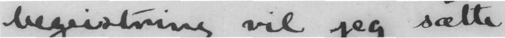begeistring vil jeg sætte
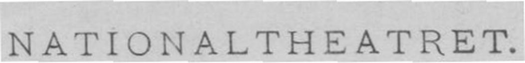NATIONALTHEATRET
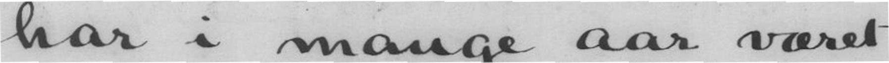har i mange aar været
